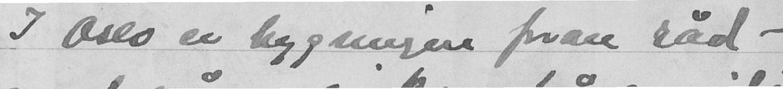I Oslo er bygningen foran råd-
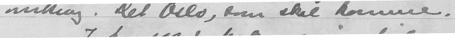omkring. Det Oslo, som skal komme.
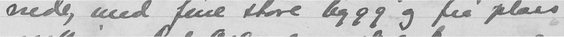nede, med fine store bygg og fri plass
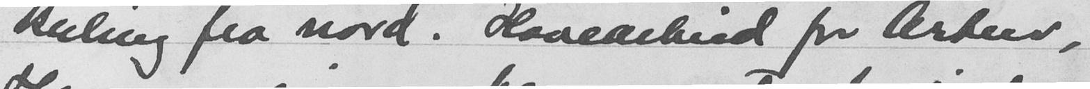kuling fra nord. Havearbeid for Astrid,
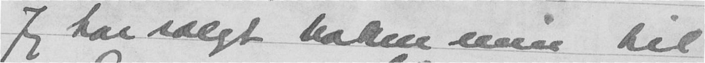Jeg har solgt boken men til
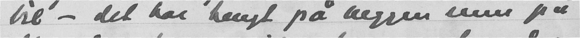til - det har hengt på veggen min på
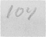104
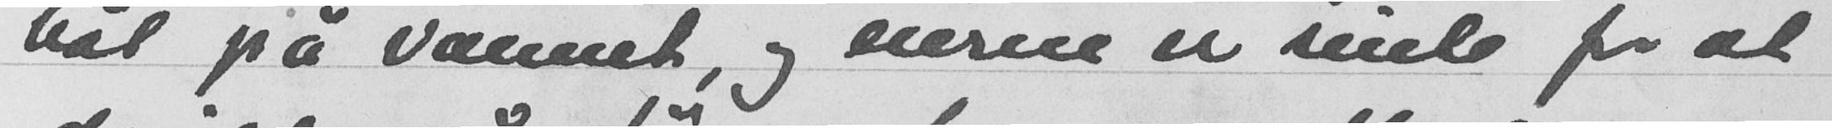båt på vannet, og eierne er sinte for at
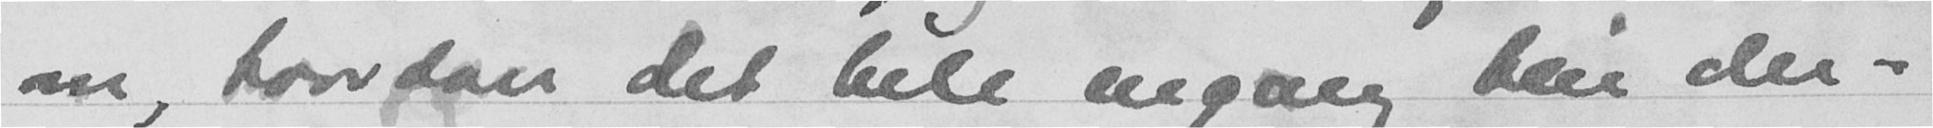om, hvordan det hele engang blir der-
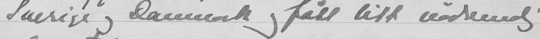Sverige og Danmark og fatt litt nødvendig
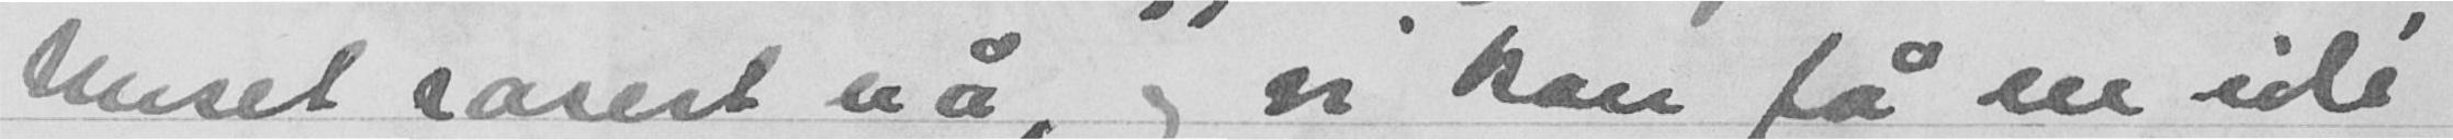huset rasert nå, og vi kan få en idé
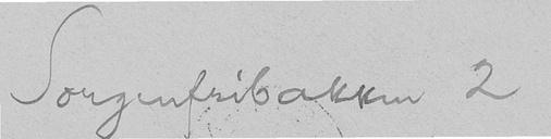Sorgenfribakken 2
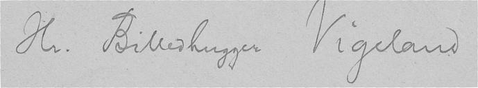Hr. Billedhugger Vigeland
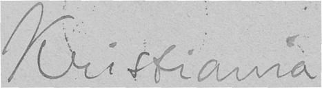Kristiania
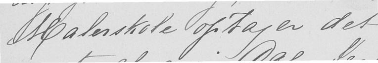Malerskole optager det
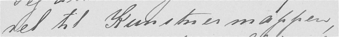rel til Kunstnermappen,
